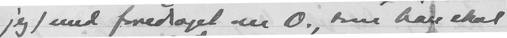jeg) med foredraget om O., som han skal
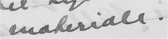materiale.
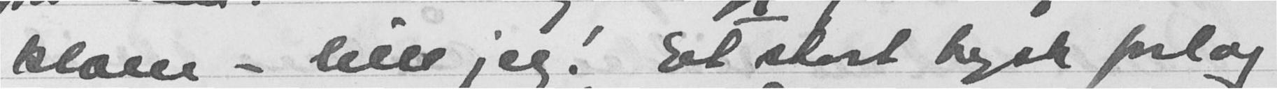kloen - lille jeg! Et stort tysk forlag
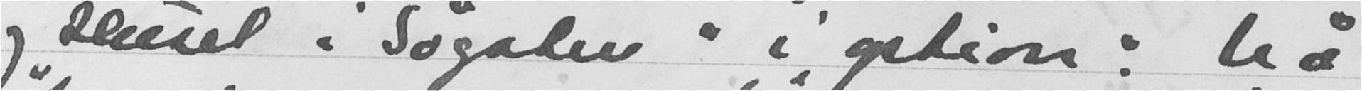og "Huset i Søgaten" i option: Nå
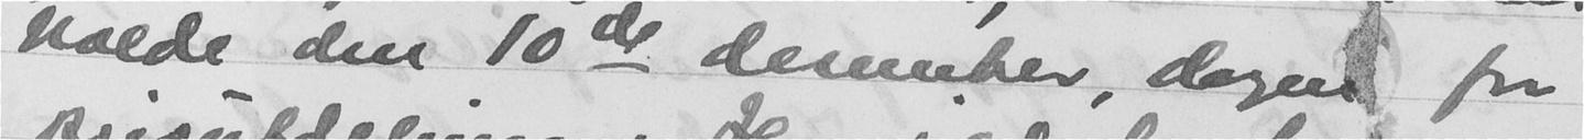holde den 10de desember, dagen før
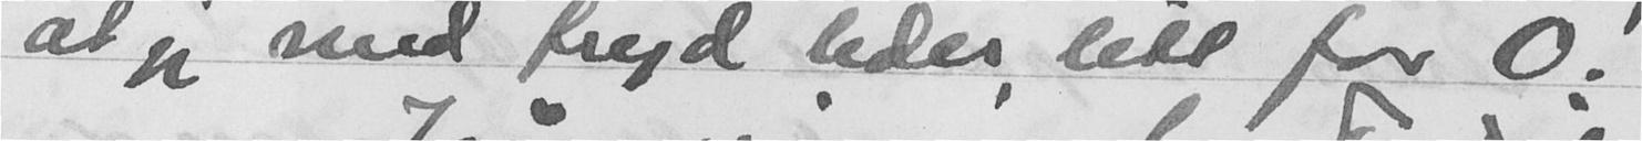at jeg med fryd lider litt for O.!
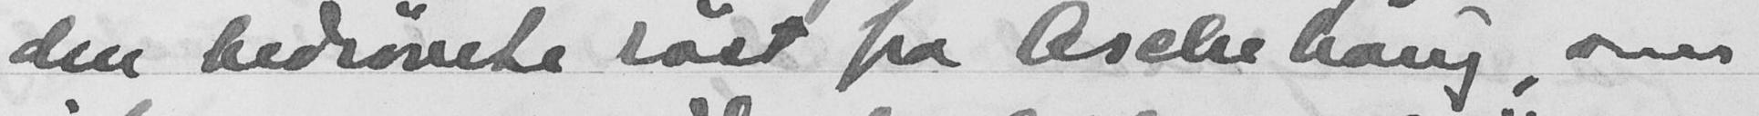den bedrøvete røst fra Aschehaug, som
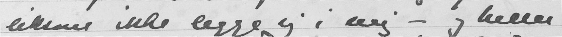liksom ikke legge sig i mig - og heller
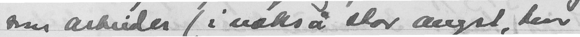som arbeider (i nokså stor angst, tror
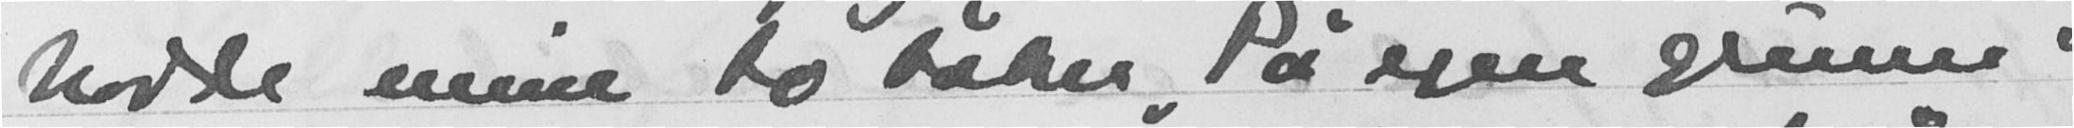hadde mine to bøker "På egen grunn"
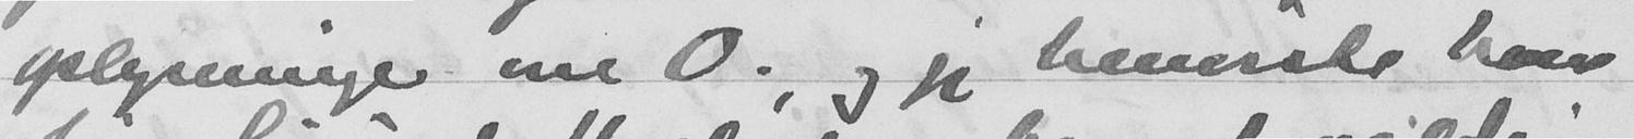oplysninger om O. og jeg henviste han
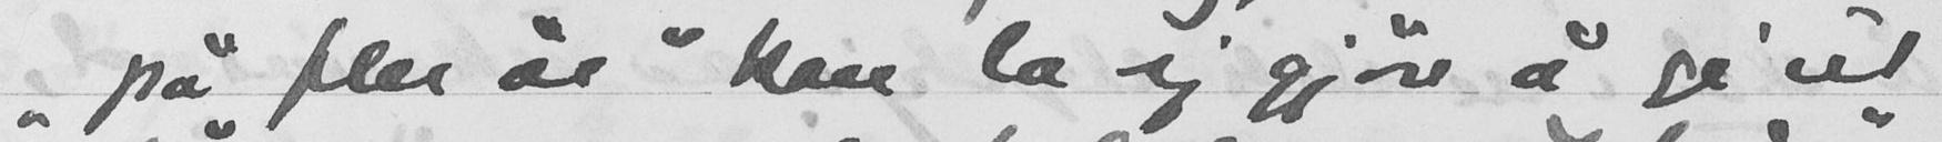"på fler år" kan la sig gjøre å gi ut
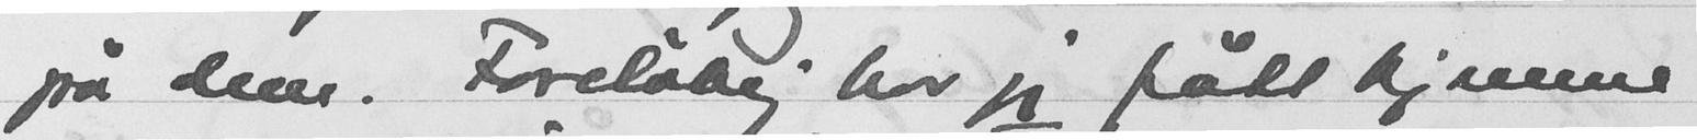på dem. Foreløbig har jeg fått kjenne
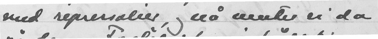med repressalier, og nå venter vi da
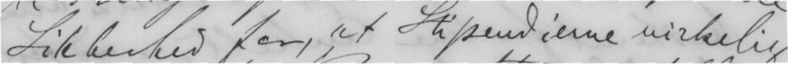Sikkerhed for, at Stipendierne virkelig
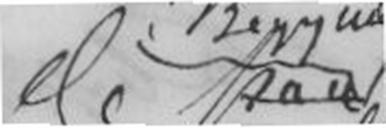de paa i
